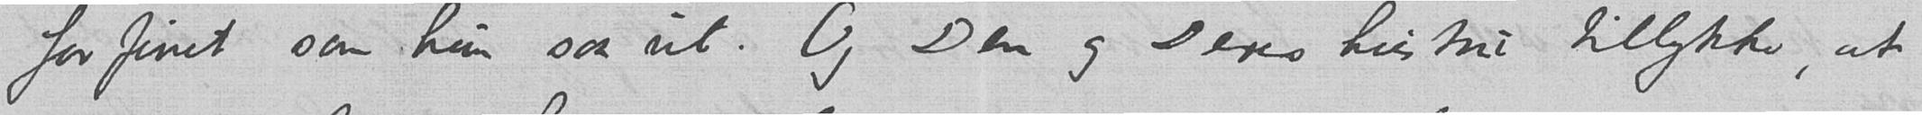forfinet som hun saa ut. Og Dem og Deres hustru tillykke, at
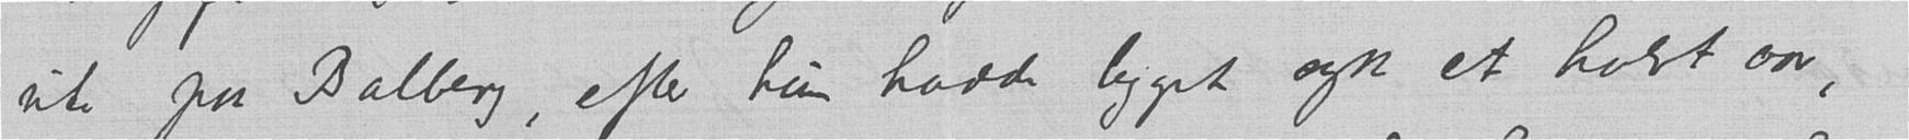ute paa Balberg, efter hun hadde ligget syk et halvt aar,
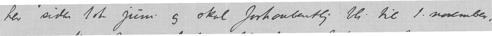her siden 1ste juni og skal forhaabentlig bli til 1. november,
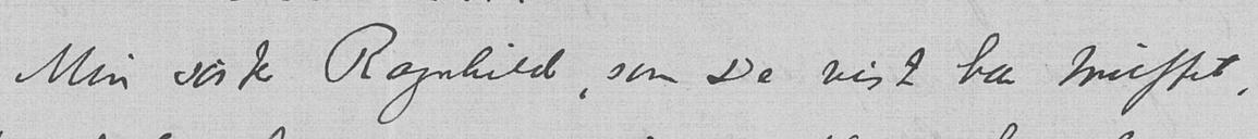Min søster Ragnhild, som De vist har truffet,
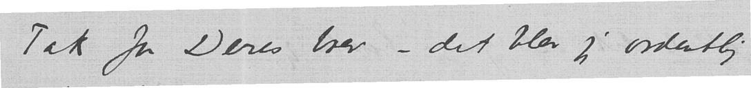Tak for Deres brev – det blev jeg ordentlig
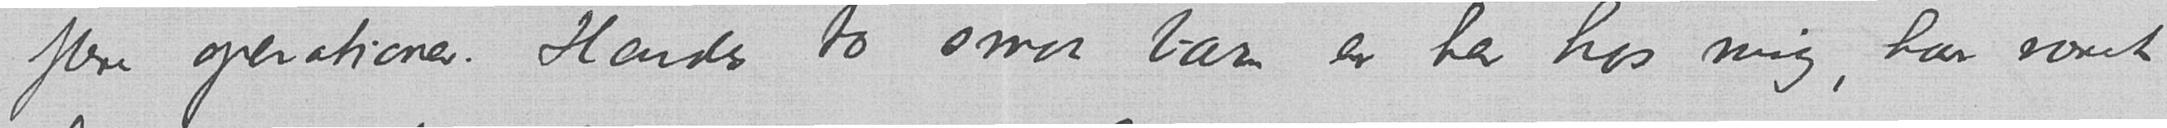flere operationer. Hendes to smaa barn er her hos mig, har været
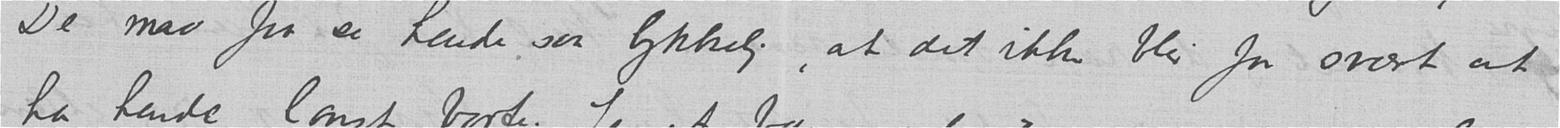De maa faa se hende saa lykkelig, at det ikke blir for svært at
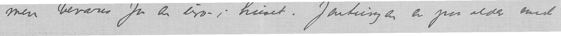men bevares for en uro – i huset. Jentungen er paa alder med
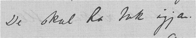De skal ha tak igjen.
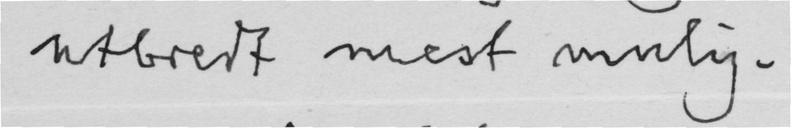utbredt mest mulig.
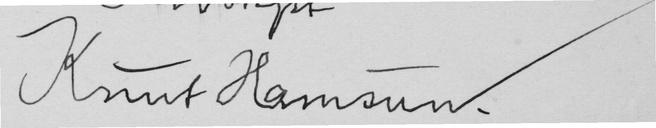Knut Hamsun.
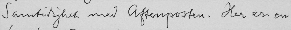Samtidighet med Aftenposten. Her er en
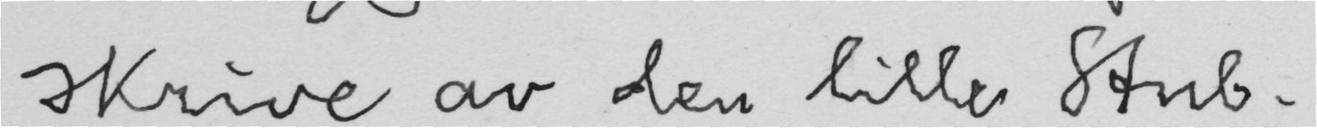skrive av den lille Stub.
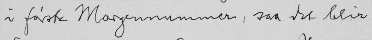i første Morgennummer, saa det blir
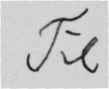Til
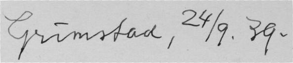Grimstad, 24/9. 39.
